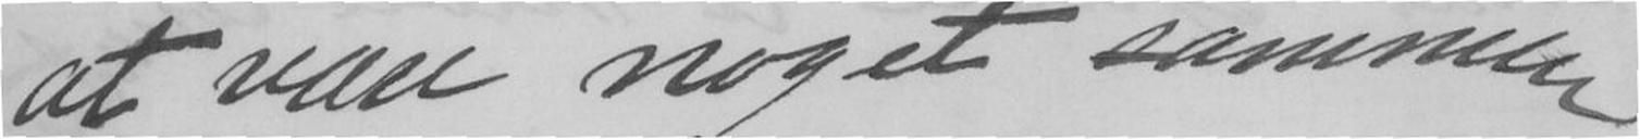at være noget sammen
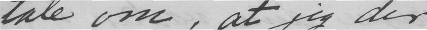tale om, at jeg der
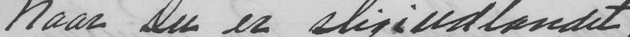Naar Du er slig i udlandet,
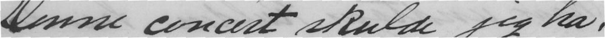Denne concert skulde jeg ha'
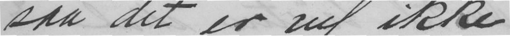saa det er vel ikke
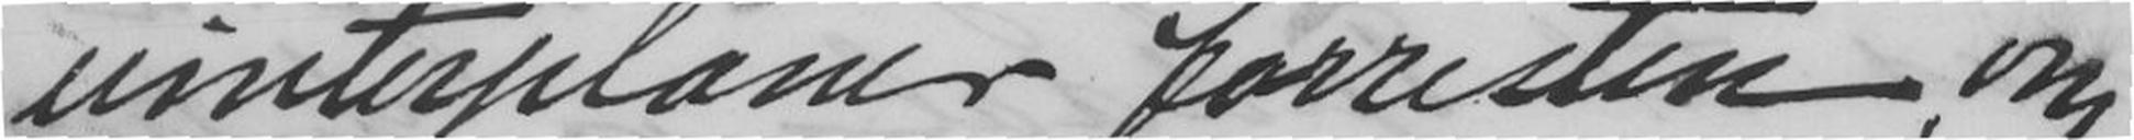vinterplaner forresten, om
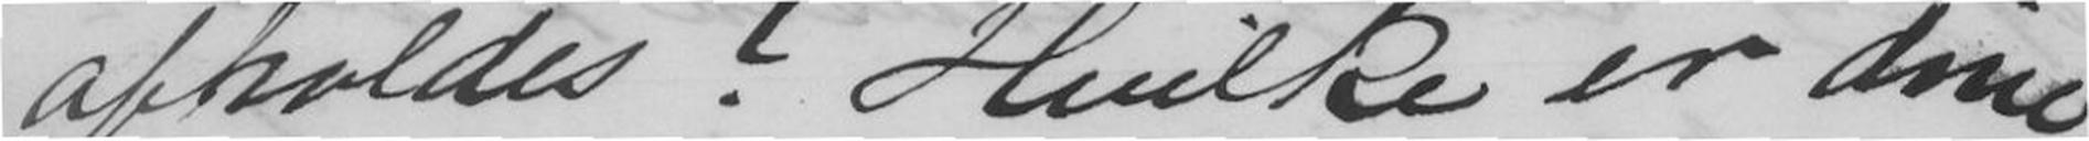afholdes? Hvilke er dine
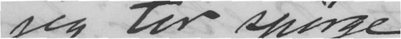jeg tør spørge
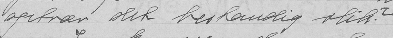optrær det bestandig slik?
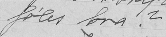føles bra?
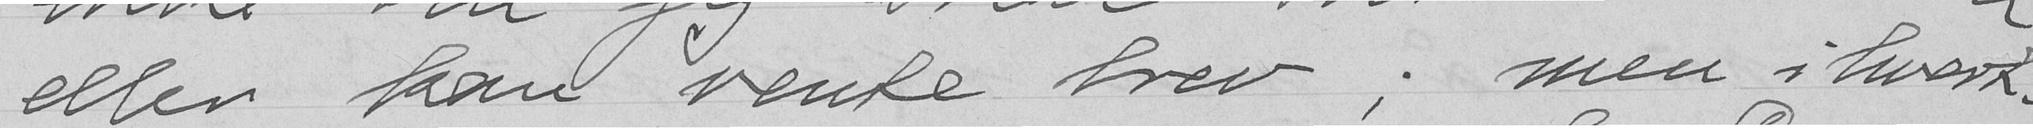eller kan vente brev; men i hvert
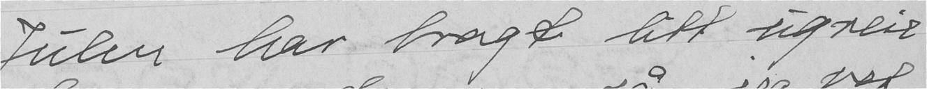Julen har bragt litt ugreie
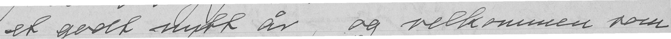et godt nytt år, og velkommen som
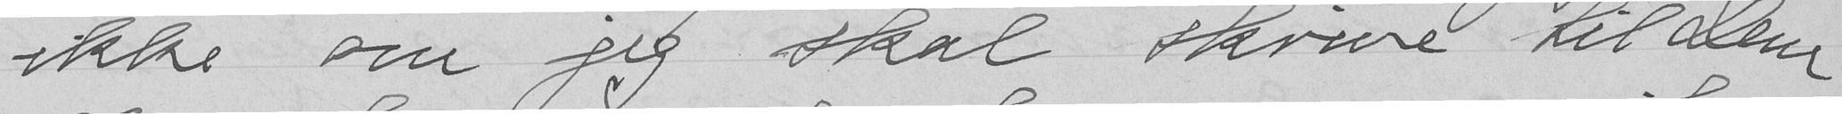ikke om jeg skal skrive til Dem
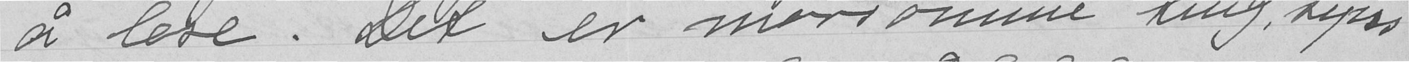å lese. Det er morsomme ting, synes
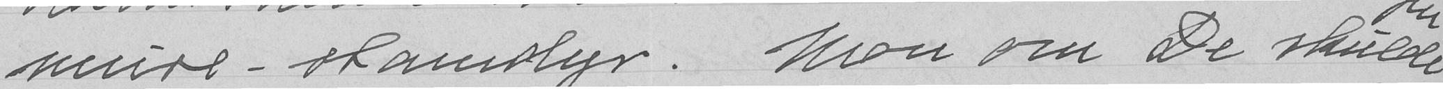muse-stamdyr. Mon om De skulde
